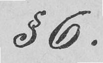§ 6.
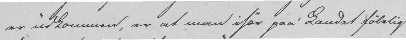er udkommen, er at man især paa Landet følelig
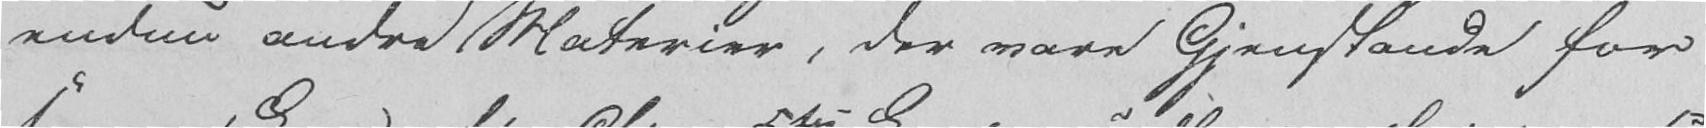endnu andre Materier, der vare Gjenstande for
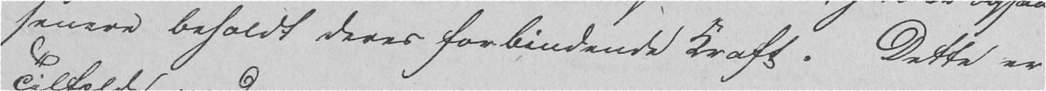senere beholdt deres forbindende Kraft. Dette er
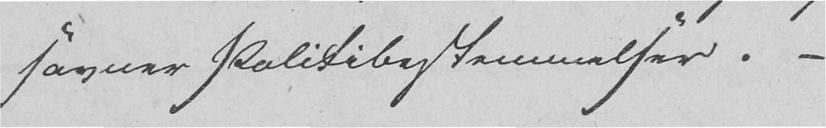savner Politibestemmelser. -
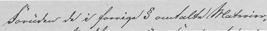Foruden de i forrige § omtalte Materier,
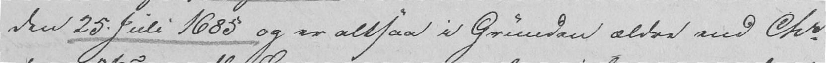den 25. Juli 1685 og er altsaa i Grunden ældre end Chr
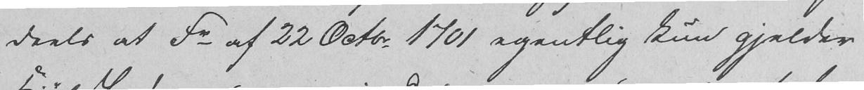deels at Fr af 22 Octbr. 1701 egentlig kun gjelder
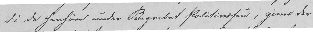di de henhøre under Begrebet Politivæsen, gives der
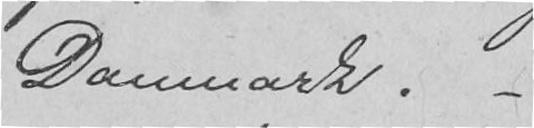Danmark. -
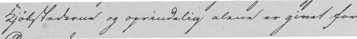Kjøbstederne og oprindelig alene er givet for
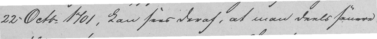22. Octbr 1701, kan sees deraf, at man deels senere
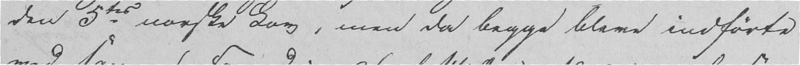den 5tes norske Lov, men da begge bleve indførte
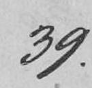39.
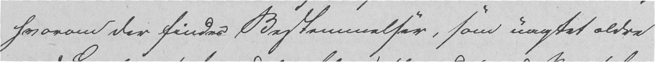hvorom der findes Bestemmelser, som uagtet ældre
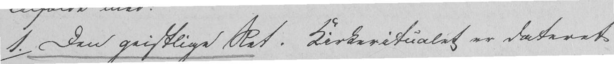1. Den geistlige Ret. Kirkeritualet er dateret
